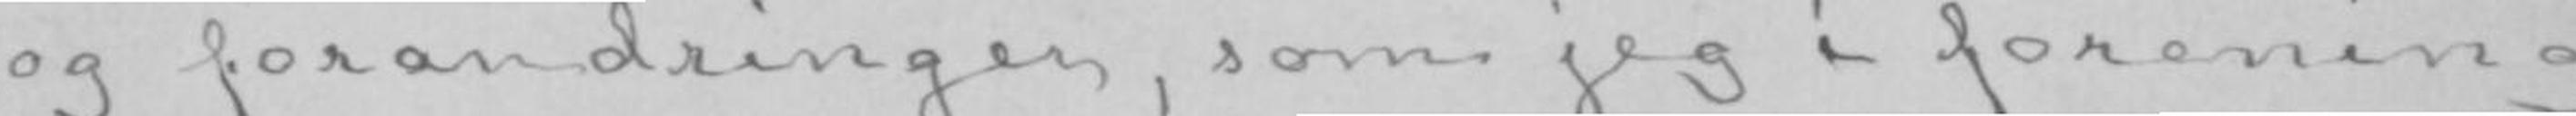og forandringer, som jeg i forening
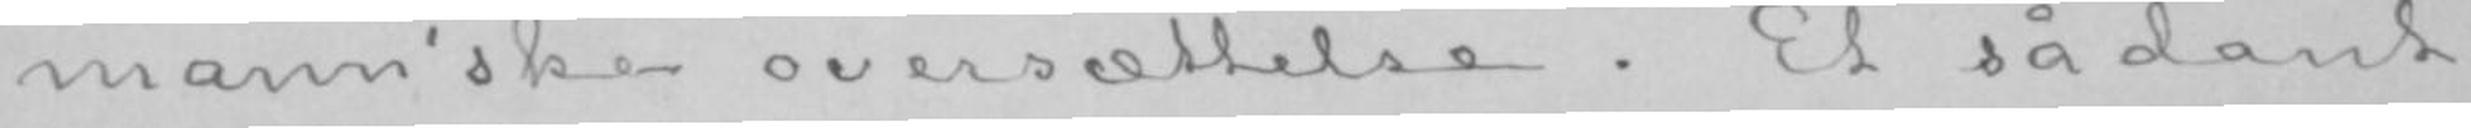mann'ske oversættelse. Et sådant
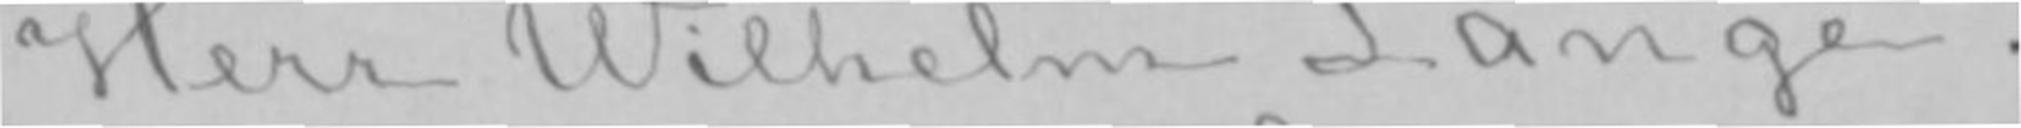Herr Wilhelm Lange.
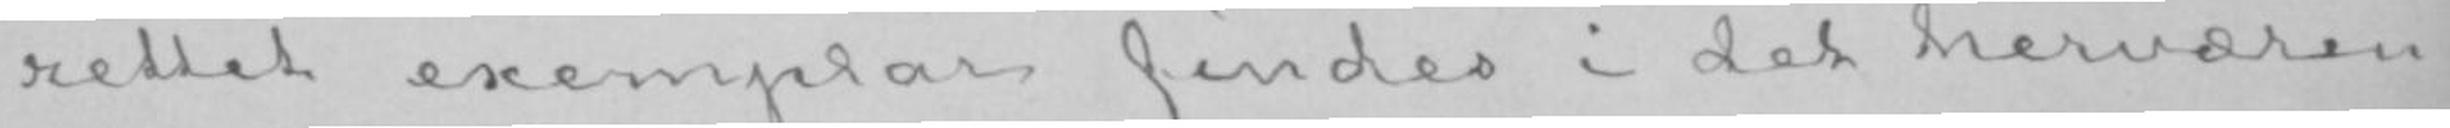rettet exemplar findes i det herværen-
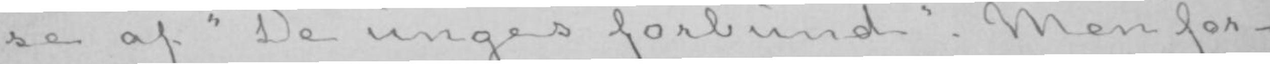se af «De unges forbund». Men for-
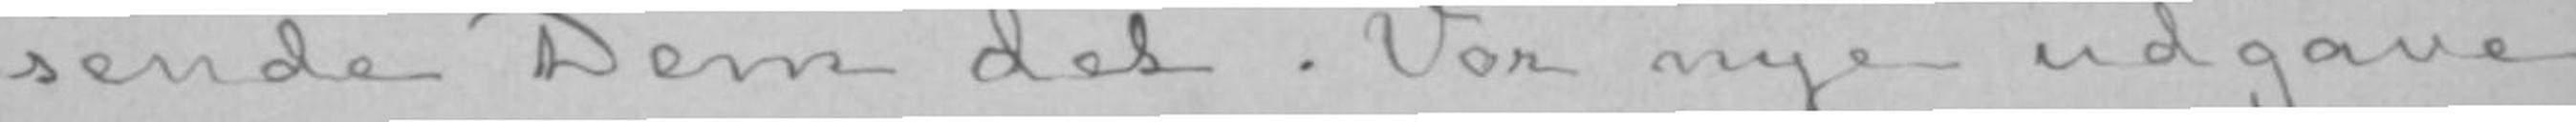sende Dem det. Vor nye udgave
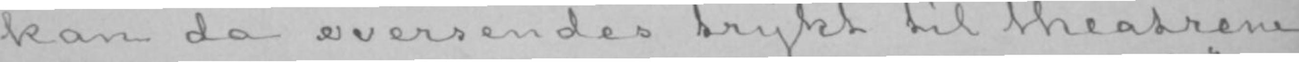kan da oversendes trykt til theatrene
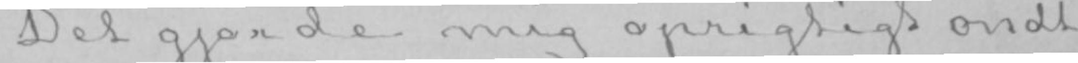Det gjorde mig oprigtigt ondt
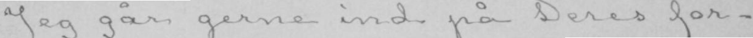Jeg går gerne ind på Deres for-
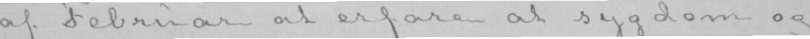af Februar at erfare at sygdom og
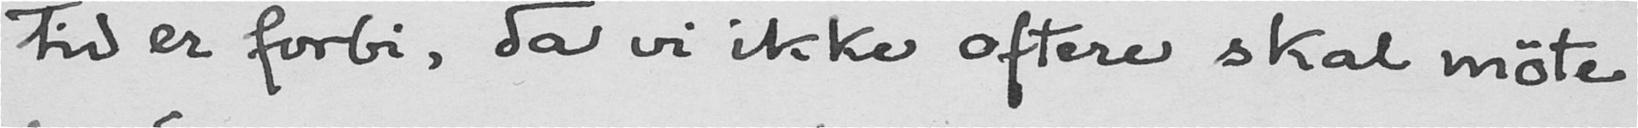tid er forbi, da vi ikke oftere skal møte
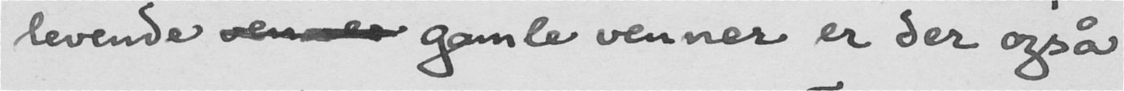levende venner gamle venner er der også
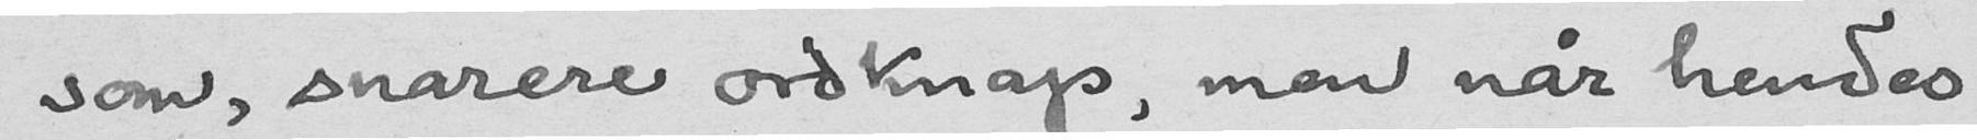som, snarere ordknap, men når hendes
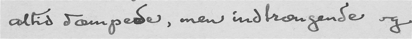altid dæmpede, men indtrængende og
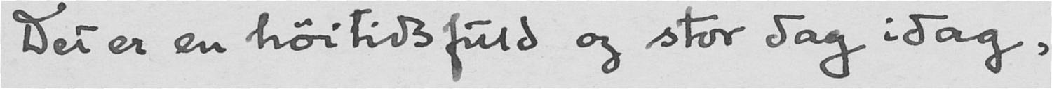Det er en høitidsfuld og stor dag idag,
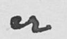er
